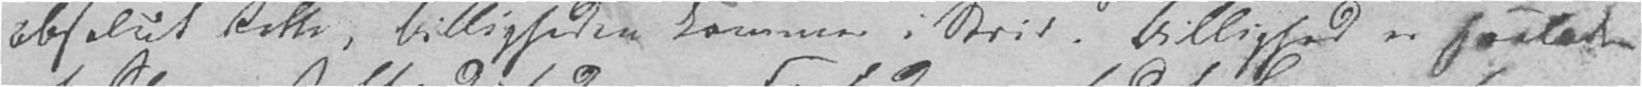absolut Rette, Billigheden kommer i Strid. Billighed er saaledes
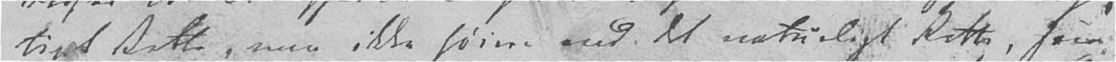tivt Rette, men ikke høiere end det naturligt Rette, som
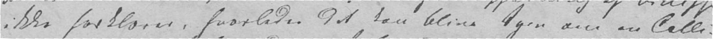ikke forklarer, hvorledes det kan blive Spm om en Colli-
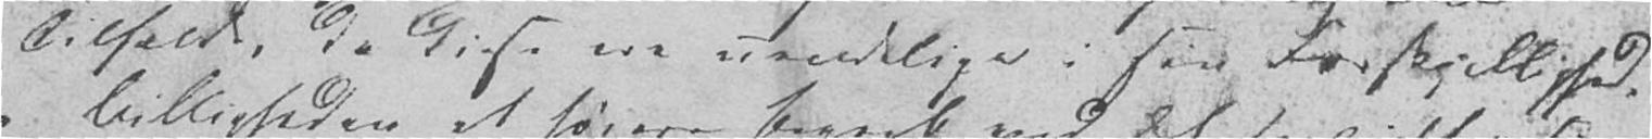Tilfælde, da disse ere uendelige i sin Forskjellighed.
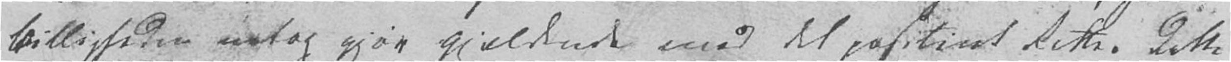Billigheden netop gjør gjældende mod det positivt Rette. Dette
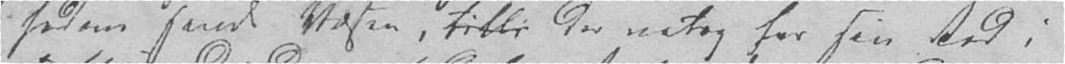hedens sande Væsen, tilli der netop har sin Rod i
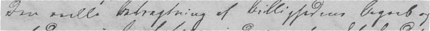Den reelle Betragtning af Billighedens Begreb og
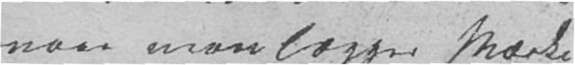naar man lægger Mærke
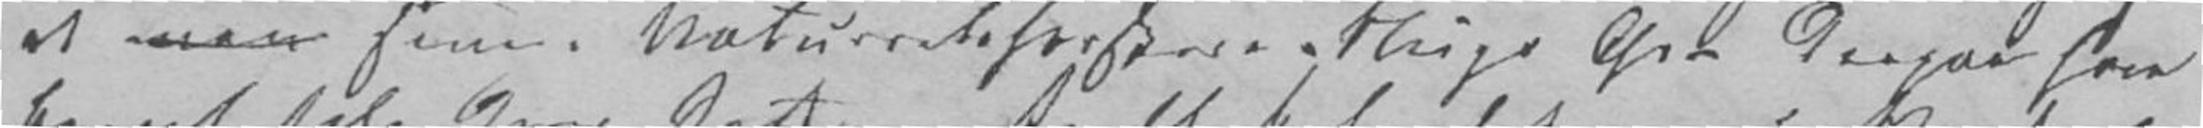at man senere Naturretsforskere slugs Grad derpaa have
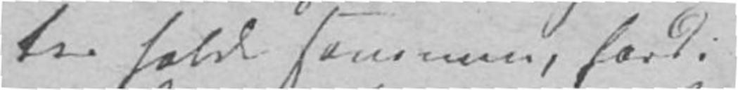ter falde sammen, fordi
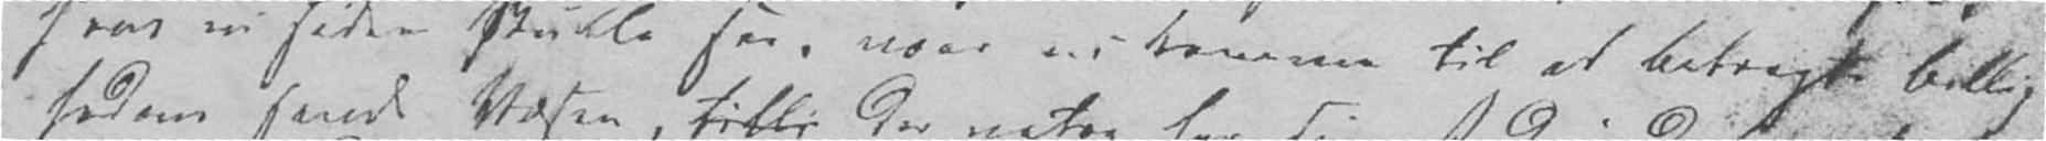som vi siden skulle see, naar vi kommer til at betragte Billig-
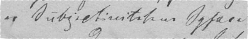er Subjectivitetens Sphære.
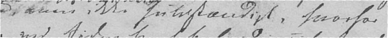men ikke fuldstændigt, hvorfor
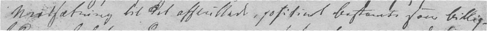i Modsætning til det afsluttede, positivt Bestemte som Billig-
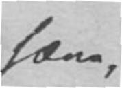hæve,
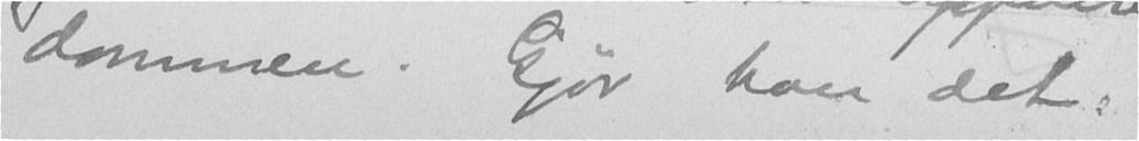dommen. Gjør han det
